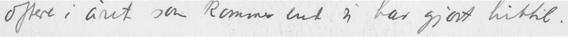oftere i året som kommer end vi har gjort hittil.
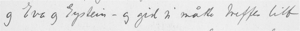og Eva og Eystein - og gid vi måtte treffes litt
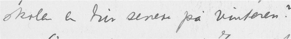skolen en tur senere på vinteren?
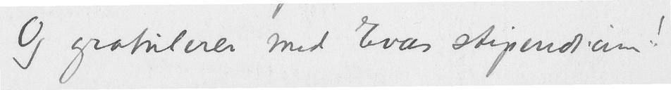Og gratulerer med Evas stipendium!
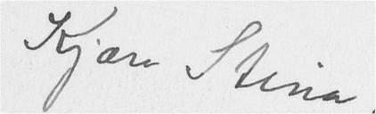Kjære Stina,
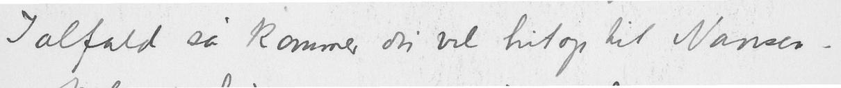Ialfald så kommer du vel hitop til Nansen-
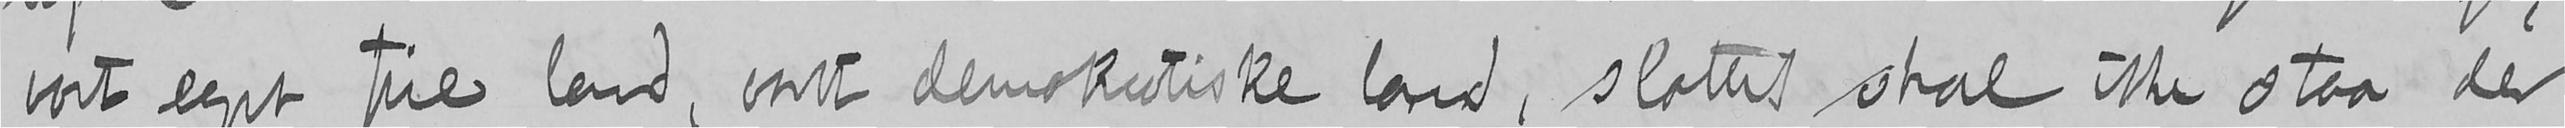vort eget frie land, vort demokratiske land, slottet skal ikke staa der
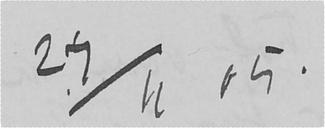27/11 05.
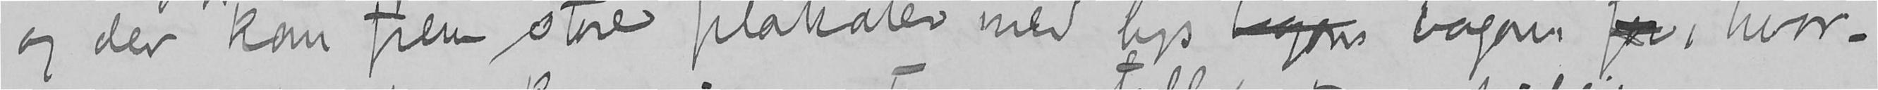og der kom frem store plakater med lys bagom bagom for, hvor-
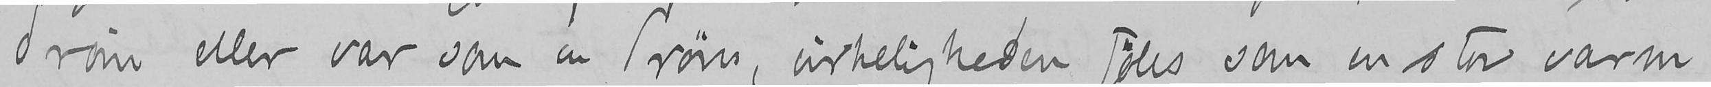drøm eller var som en drøm, virkeligheden føles som en stor varm
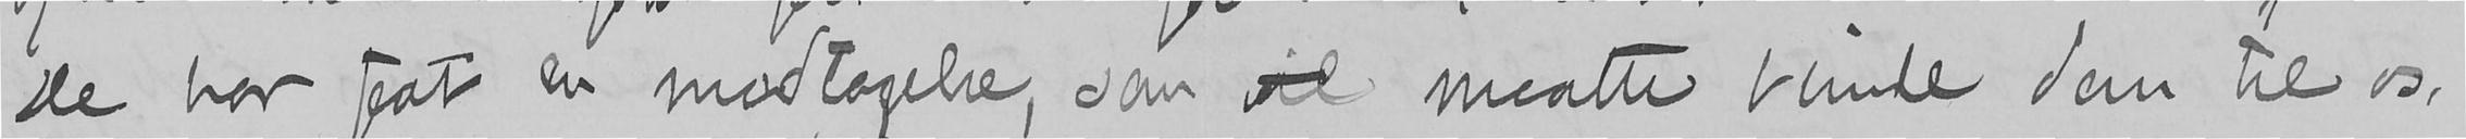De har faat en modtagelse, som vil maatte binde dem til os,
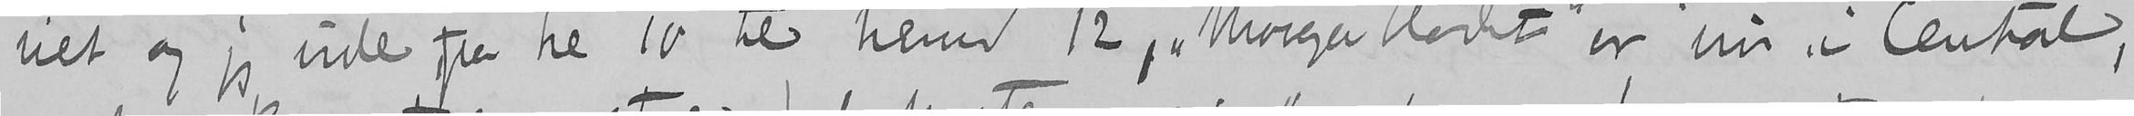riet og jeg ude fra kl 10 til henmod 12, "Morgenbladet" er nu i Central,
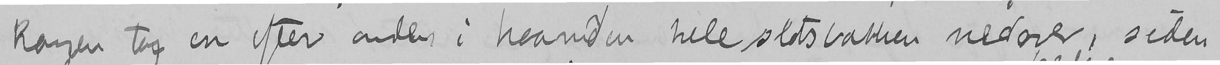kongen tog en efter anden i haanden hele slotsbakken nedover, siden
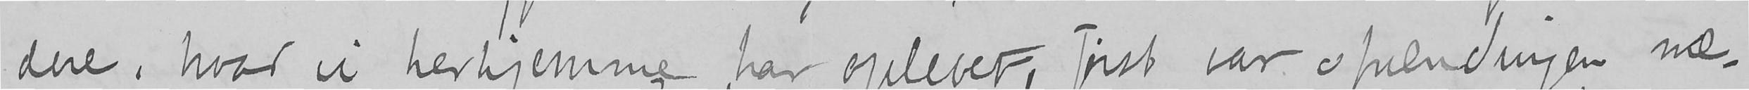dere, hvad vi herhjemme har oplevet, først var spendingen næ-
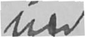ind
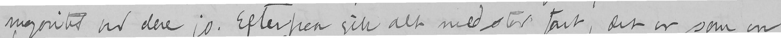majoritet ved dere jo. Efterpaa gik alt med stor fart, det er som en
